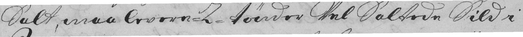Salt maa levere 2 tønder Vel Saltede Sild i
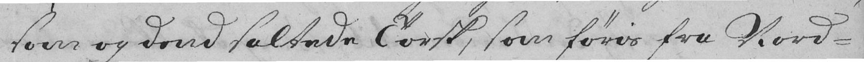som og dend saltede Torsk, som føris fra Nord-
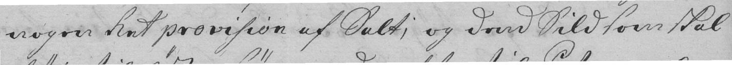nogen Ret provision af Salt, og dend Sild som skal
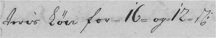teris Løn for 16 og 12 sk.
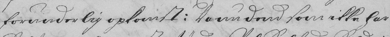forunderlig opkomst: Da nu dend som ikke har
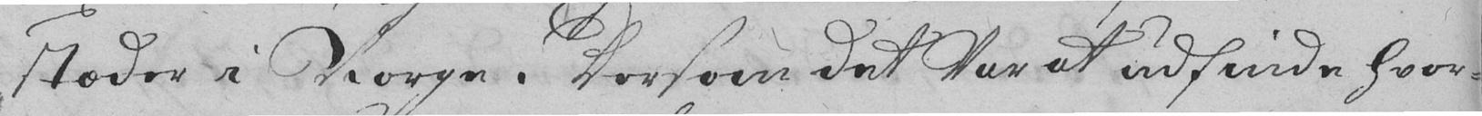stæder i Norge. Dersom det var at udfinde hvor-
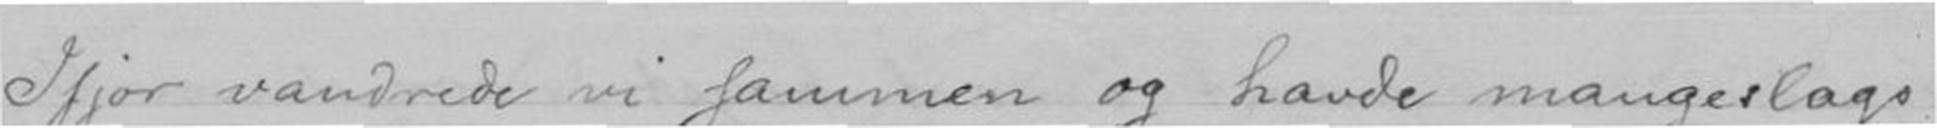Ifjor vandrere vi sammen og havde mangeslags
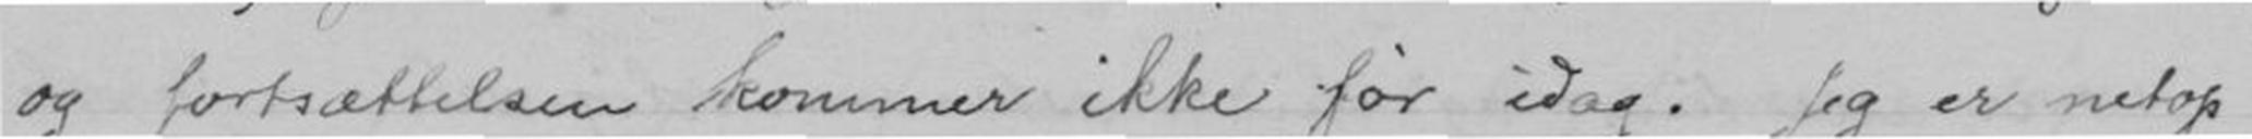og fortsættelsen kommer ikke før idag. Jeg er netop
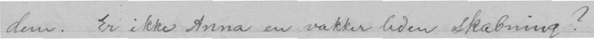dem. Er ikke Anna en vakker liden skabning?
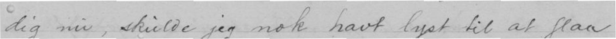dig nu, skulde jeg nok havt lyst til st slaa
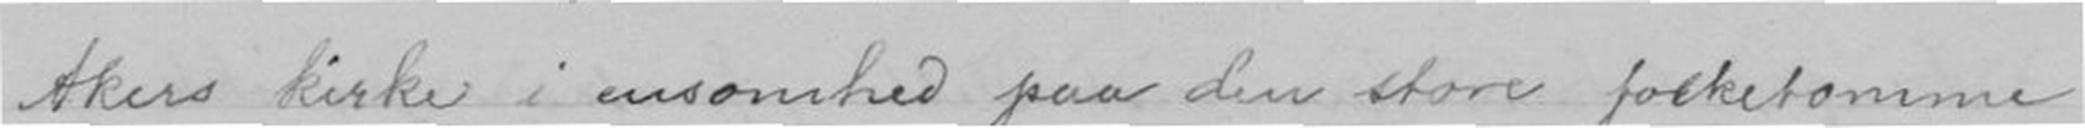Akers kirke i ensomhed paa den store folketomme
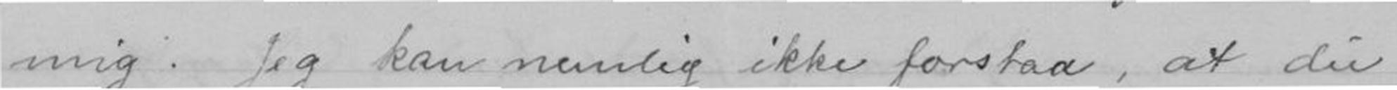mig. Jeg kan nemlig ikke forstaa, at du
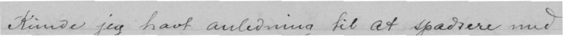Kunde jeg havt andledning til at spadsere med
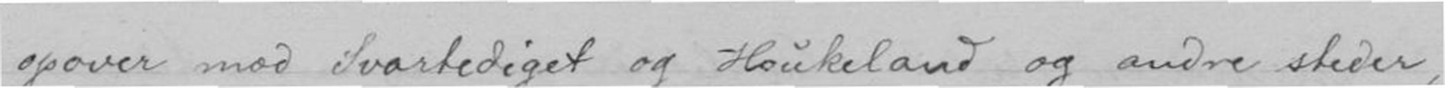opover mod Svartediget og Haukeland og andre steder,
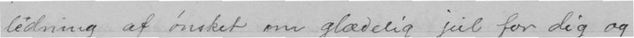ledning af ønsket om glædelig jul for dig og
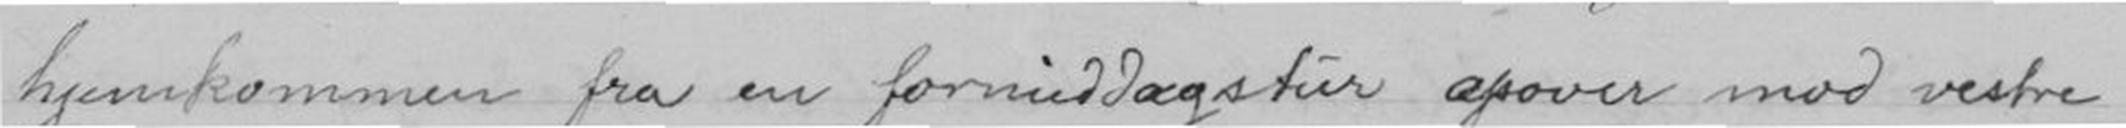hjemkommen fra en formiddagstur opover mod vestre
